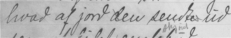hvad af jord den sendte ud
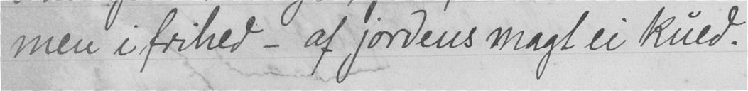men i frihed - af jordens magt ei kued.
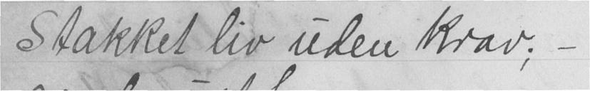Stakket liv uden krav; -
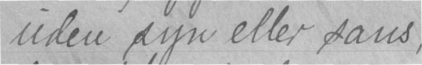uden syn eller sans,
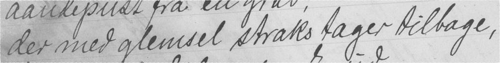der med glemsel straks tager tilbage,
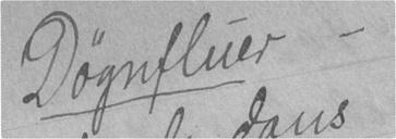Døgnfluer -
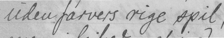uden farvers rige spil,
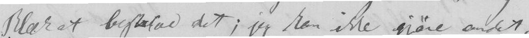Blæk at beskrive det; jeg kan ikke gjøre andet,
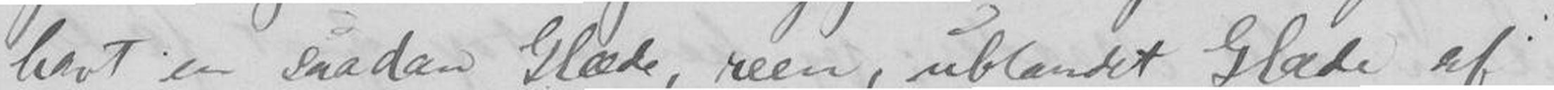havt en saadan Glæde, reen, ublandet Glæde af
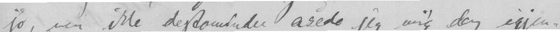jo, men ikke destomindre asede jeg mig dog igjen-
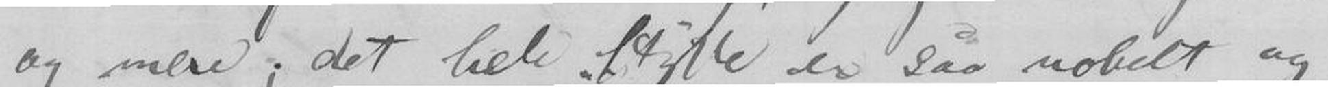og mere; det hele Stykke er saa nobelt og
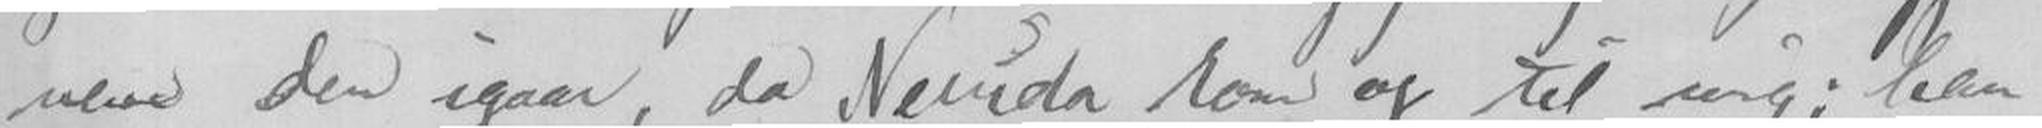nem den igaar, da Neruda kom op til mig; han
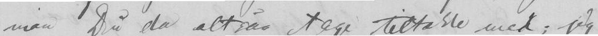maa Du da altsaa tage tiltakke med; jeg
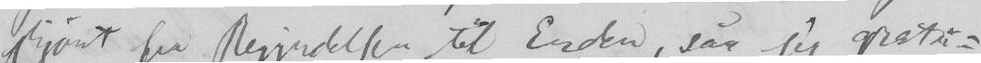skjønt fra Begyndelsen til Enden, saa jeg gratu-
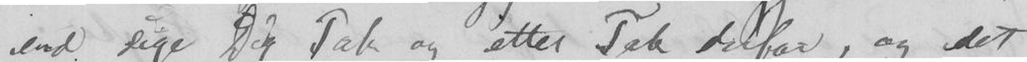and sige Dig Tak og atter Tak derfor, og det
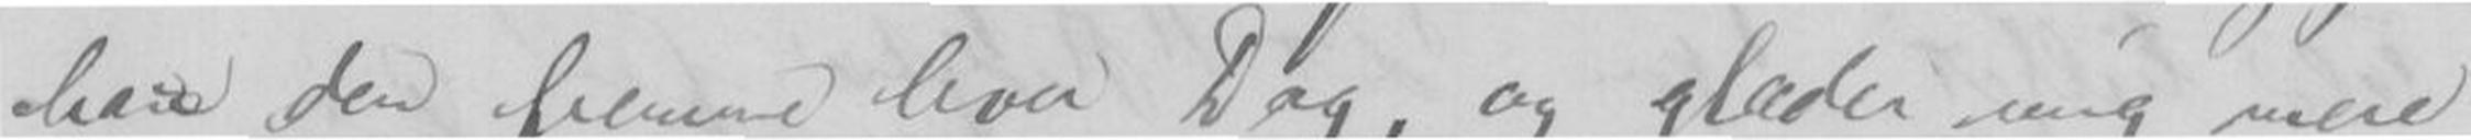har den fremme hver Dag, og glæder mig mere
